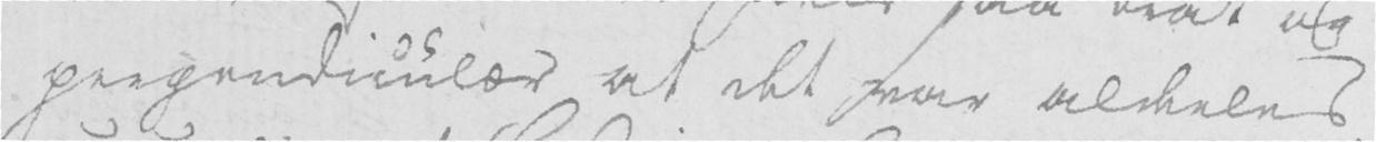perpendiculær at det var aldeles
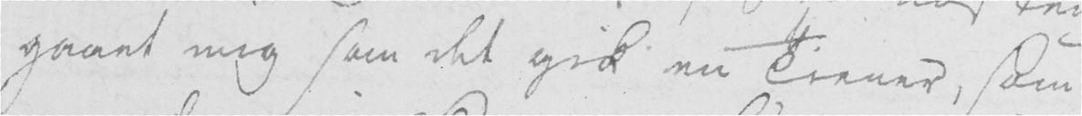gaaet mig som det gik en Tiener, som
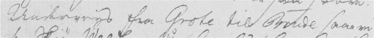Underveys fra Grote til Brude saae vi
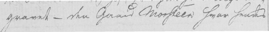gravet - Den Gaard Morsteen hvor hendes
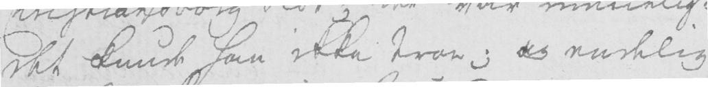det kunde han ikke troe; og endelig
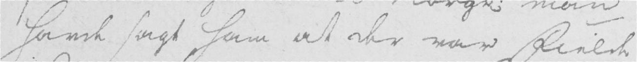havde sagt ham at der var Fielde
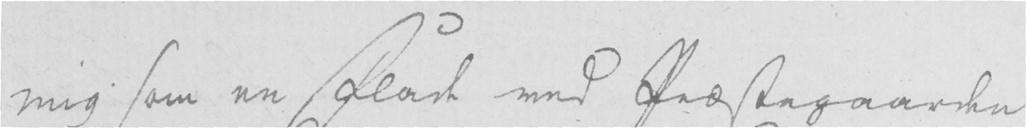mig som en Flade ved Præstegaarden
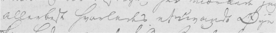allerbest hvorledes et uvandt Øye
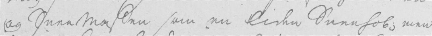og Snee Massen som en liden Sneehob; men
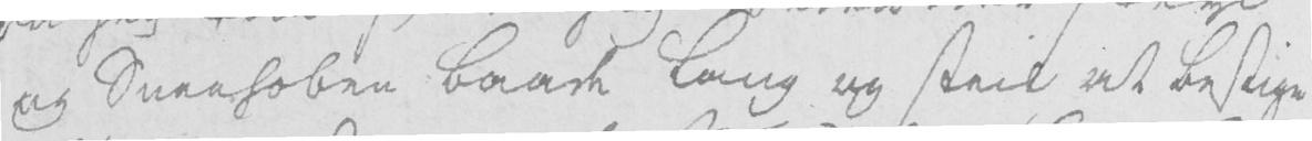og Sneehoben baade lang og steil at bestige
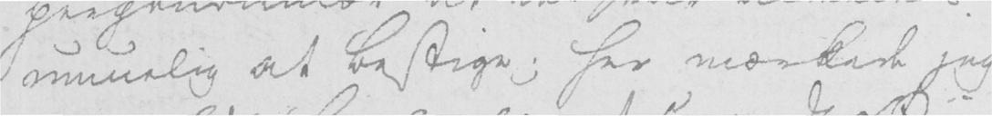umuelig at bestige; her mærkede jeg
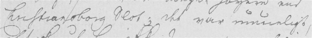Christiansborg Slot; det var umueligt,
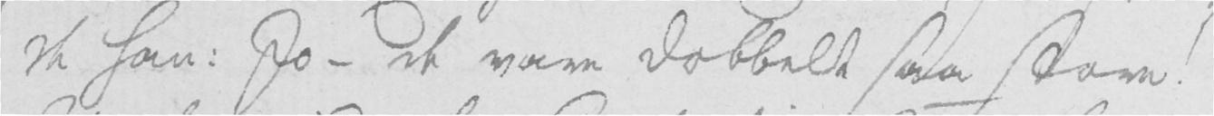de han: Jo - de vare dobbelt saa store!
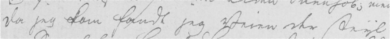da jeg kom fandt jeg Veien der steyl
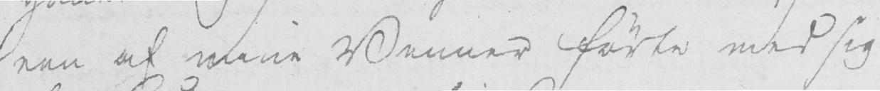een af mine Venner førte med sig
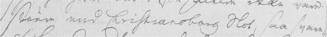større end Christiansborg Slot, saa svare-
